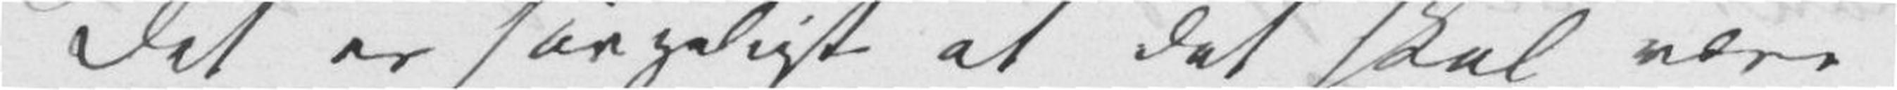Det er sørgeligt at det skal være
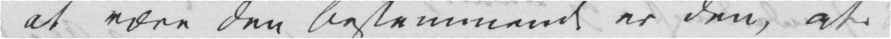at være den bestemmende er den, at
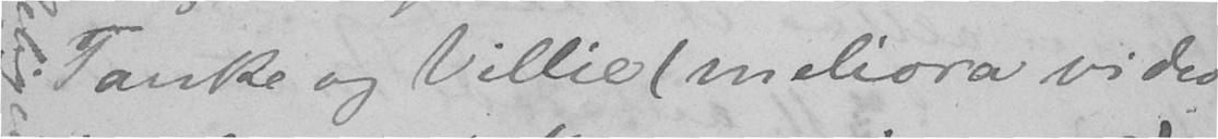Tanke og Villie (meliora vides
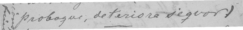proboque, deteriora sequor)
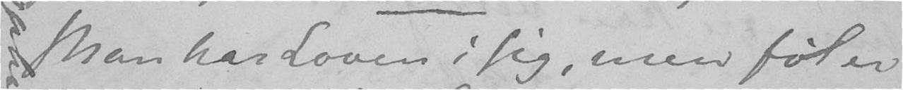Man har Loven i sig, men føler
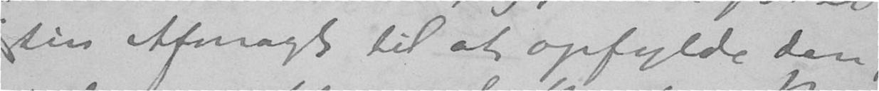sin Afmagt til at opfylde den,
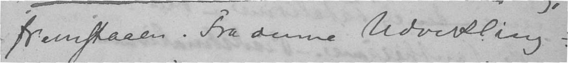fremstaaer. Fra denne Udvikling
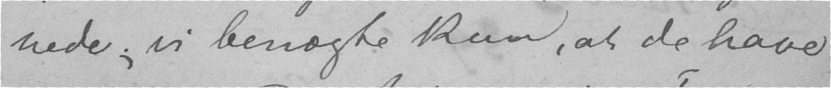nede; vi benægte kun, at de have
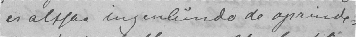er altsaa ingenlunde de oprinde-
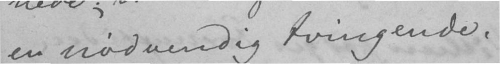en nødvendig tvingende.
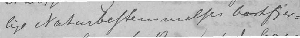lige Naturbestemmelser bortfjer-
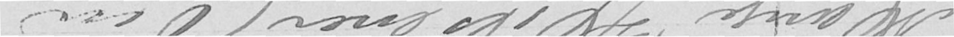Mange Hilsener Din
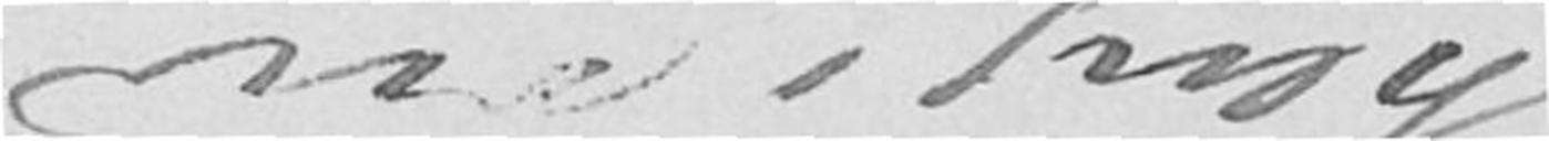hengivne
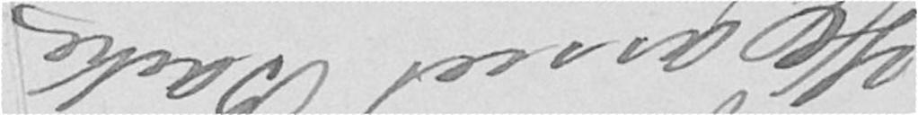Harriet Backer
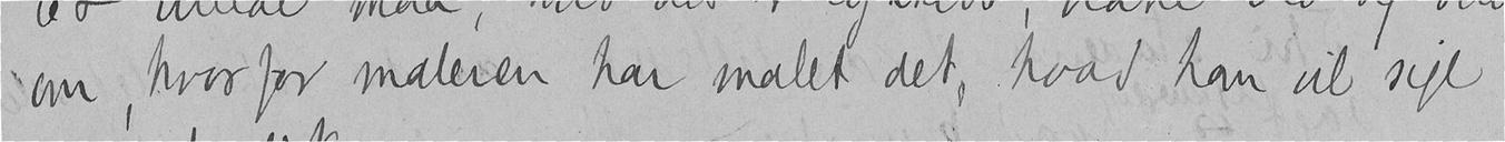om, hvorfor maleren har malet det, hvad han vil sige
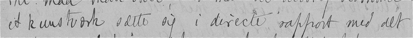et kunstværk sætte sig i directe rapport med det
